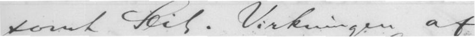somt Slid. Virkningen af
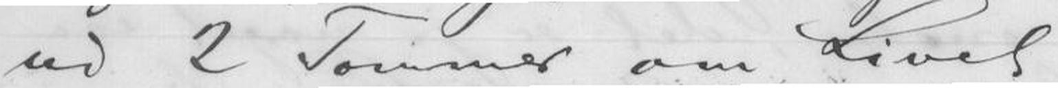ud 2 Tommer om Livet
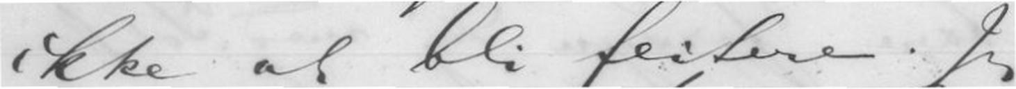ikke at bli feitere. Jeg
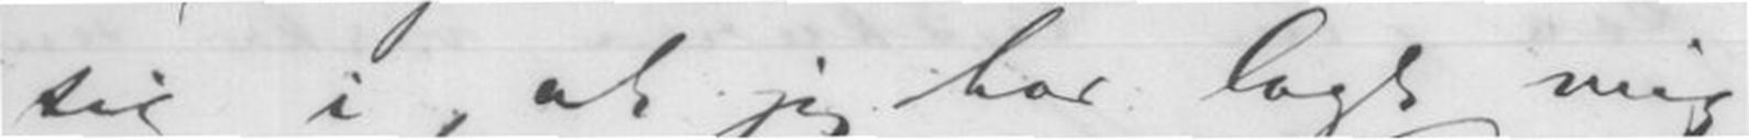sig i, at jeg har lagt mig
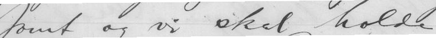somt og vi skal holde
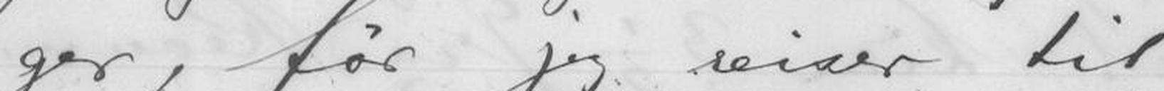ger, før jeg reiser til
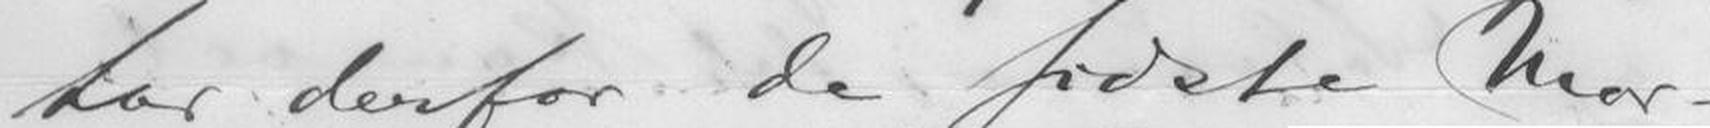har derfor de sidste Mor-
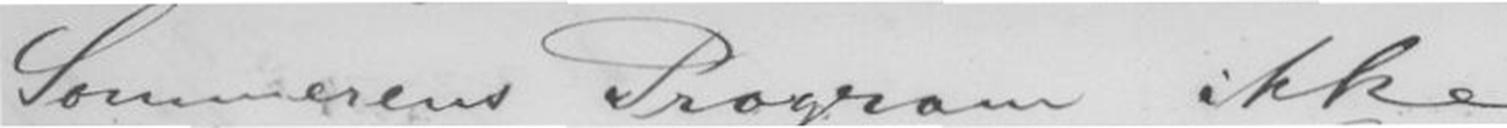Sommerens Program ikke
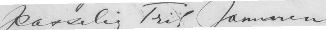passelig Trit sammen
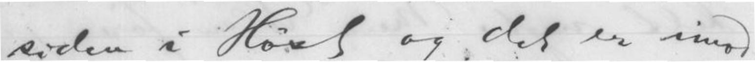siden i Høst og det er imod
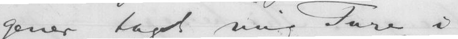gener taget mig Ture i-
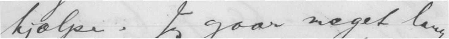hjælpe. Jeg gaar meget lang-
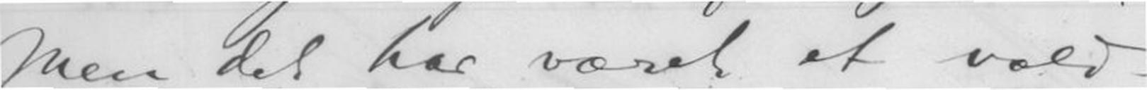Men det har været et vold-
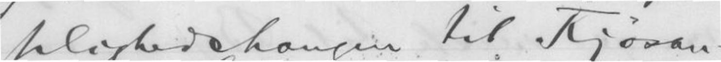salighedshaugen til Fjøsan-
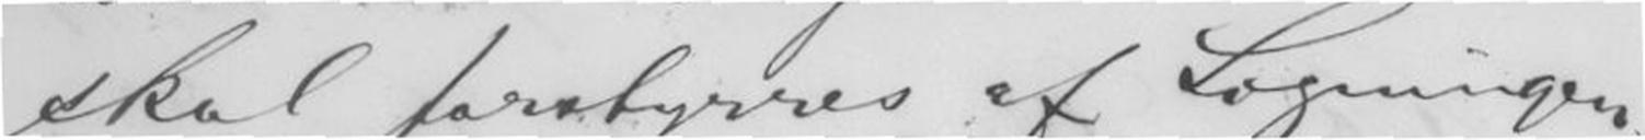skal forstyrres af Ligningen.
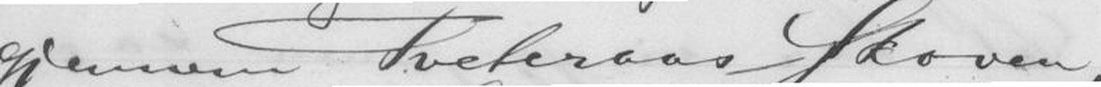gjennem Tveteraas-Skoven,
